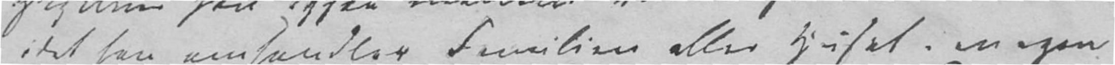idet han omhandler Familien eller Huset i en egen
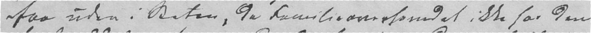faa uden i Staten, da Familieoverhovedet ikke har den
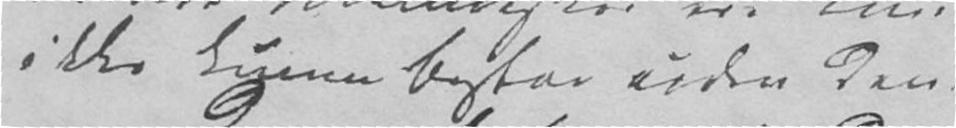ikke kunne bestaa uden den.
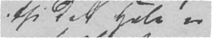thi det Hele er
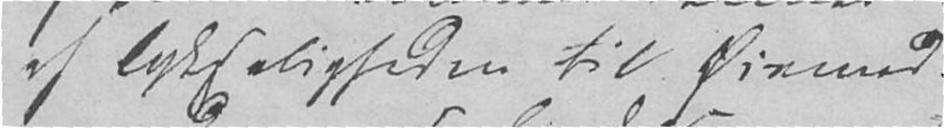af Lyksaligheden til Øiemed.
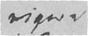rigere
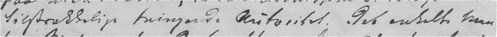tilstrækkelige tvingende Autoritet. Det enkelte Men-
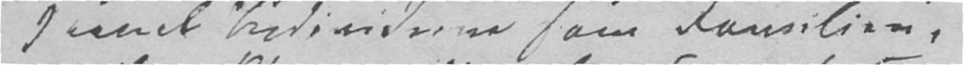saavel Individerne som Familien,
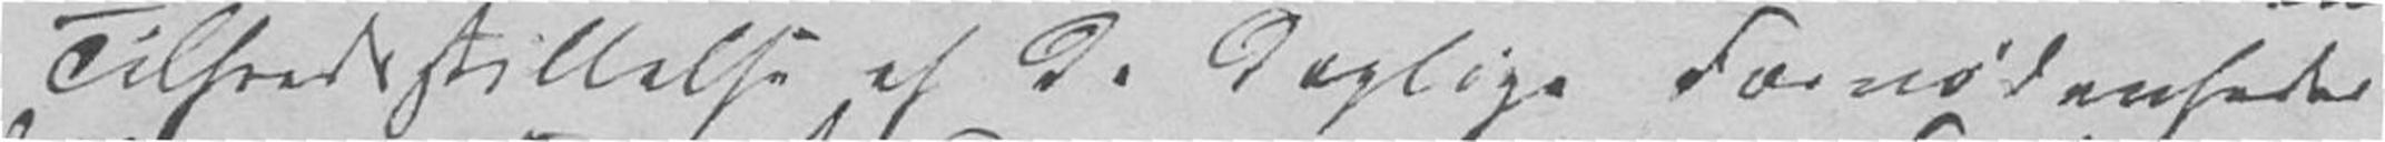Tilfredsstillelse af de daglige Fornødenheder
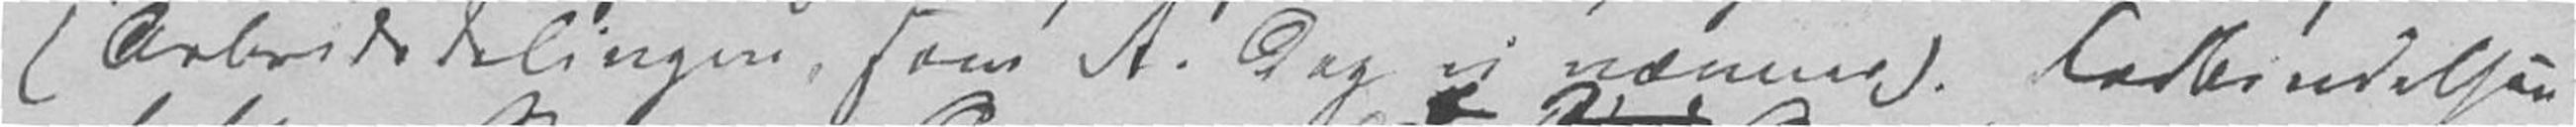(Arbeidsdelingen, som A. dog ei nævner). Forbindelsen
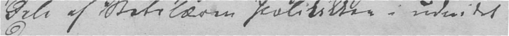Dele af Statslæren Politikken i udvidet
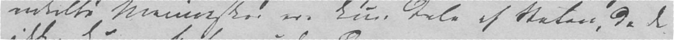enkelte Mennesker ere kun Dele af Staten, da de
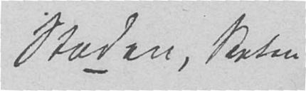Staden, Staten
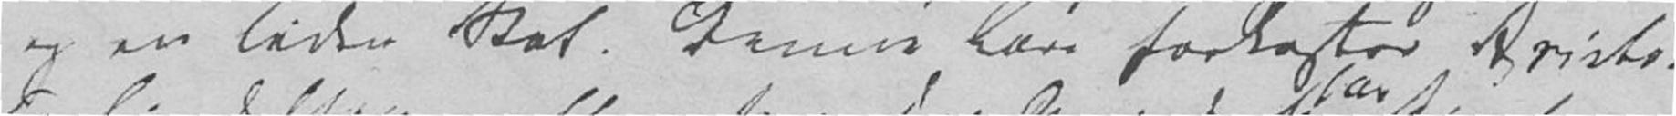og en liden Stat. Denne Lære forkaster Aristo-
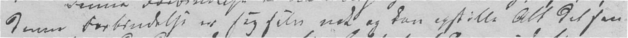denne Forbindelse er sig selv nok og kan opstille Alt det som
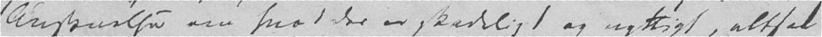Anskuelse om hvad der er skadeligt og nyttigt, altsaa
